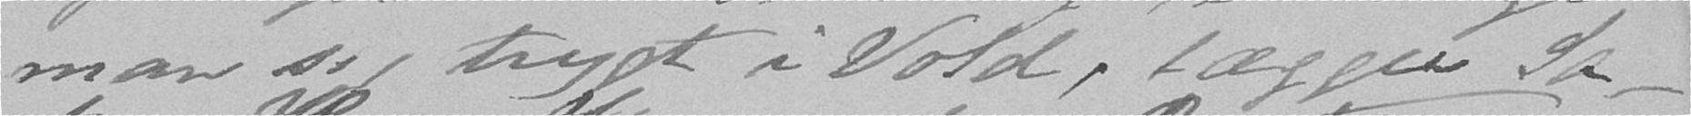man sig trygt i Vold, lægger Sa-
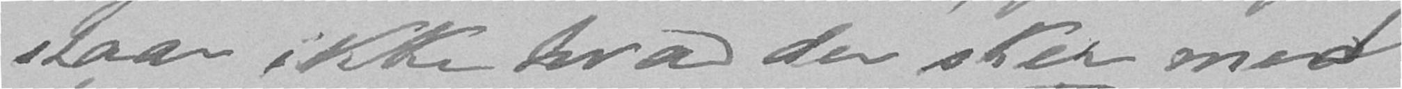staar ikke hvad der sker med
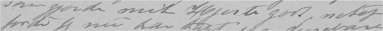som gjorde mit Hjerte godt netop
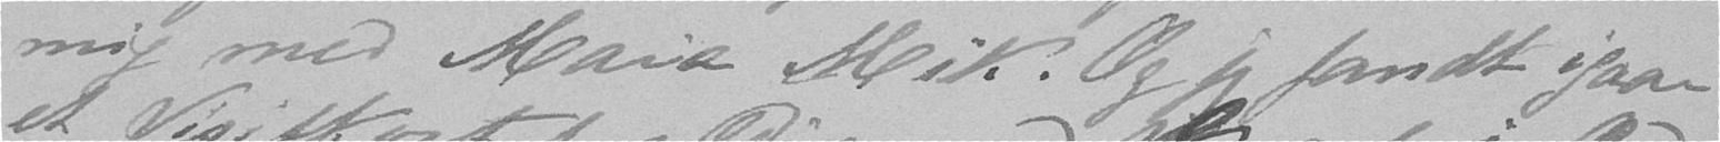mig med Maia Mik. Og jeg fandt igaar
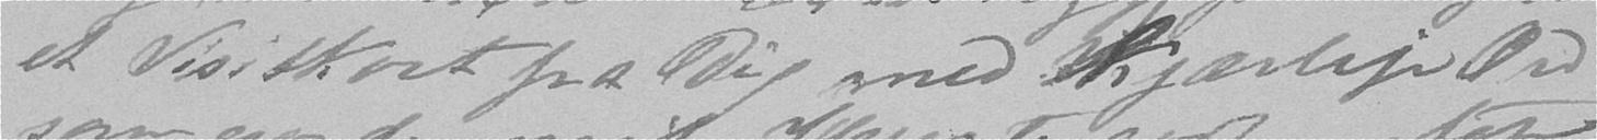et Visitkort fra Dig med kjærlige Ord
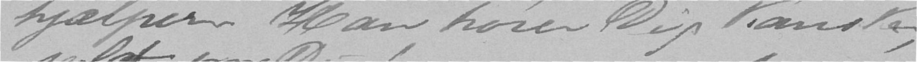hjælper. Han hører Dig kanske,
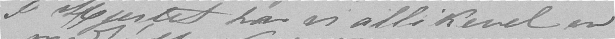I Hjertet har vi allikevel en
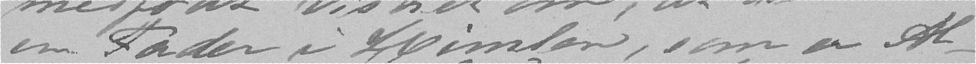en Fader i Himlen, som er Al-
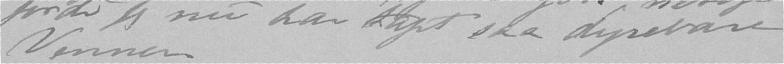fordi jeg nu har tapt saa dyrebare
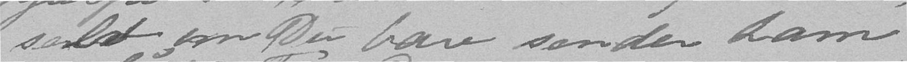selv om Du bare sender ham
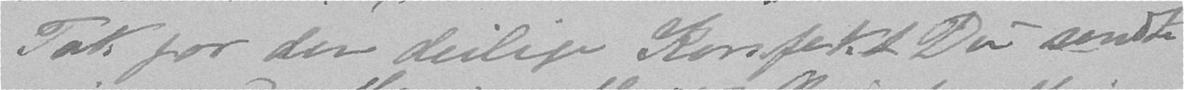Tak for den deilige Konfekt Du sendte
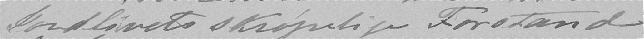Jordlivets skrøpelige Forstand.
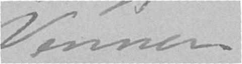Venner.
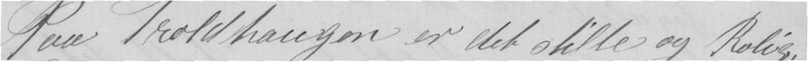Paa Troldhaugen er det stille og Roligt
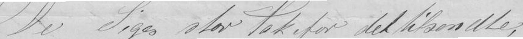De Siges stor Tak for det tilsendte,
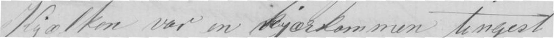Kjælken var en kjærkommen tingest
